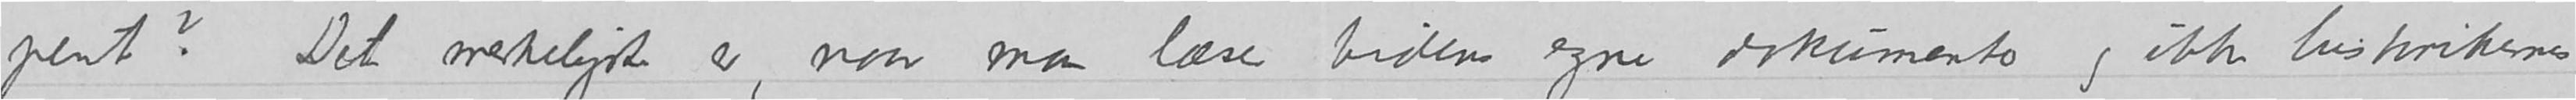pent? Det merkeligste er, naar man læser tidens egne dokumenter og ikke historikernes
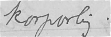korporlig.
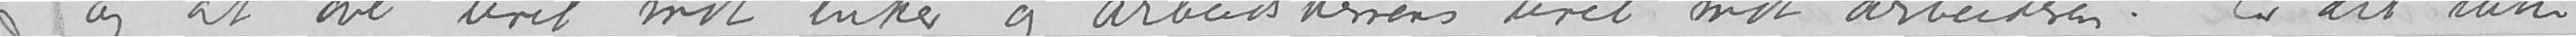og at øve uret mot enker og arbeidsherrens uret mot arbeideren! Er det ikke
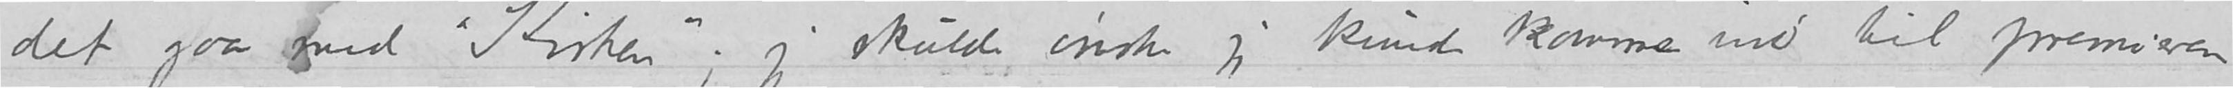det gaar med «Kirken»;  jeg skulde ønske jeg kunde komme ind til premieren.
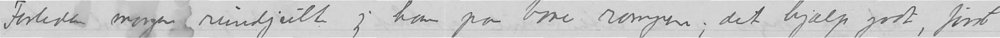Forleden morgen rundjulte jeg ham paa bare rompen; det hjalp godt, først
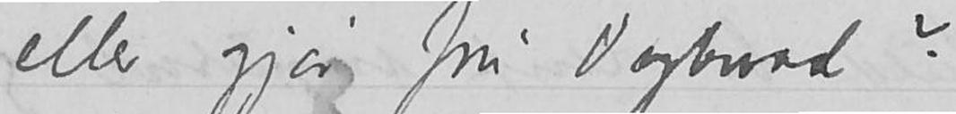eller gjør fru Dybwad?
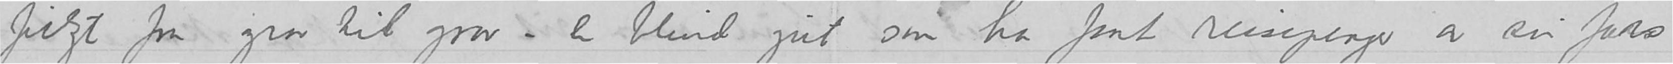fulgt fra grav til grav -en blind gut som har faat reisepenger av sin fars
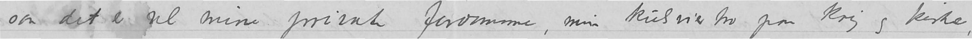saa det er vel mine private fordommer, min kulsviertro paa krig og kirke,
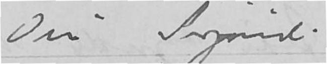Din Sigrid
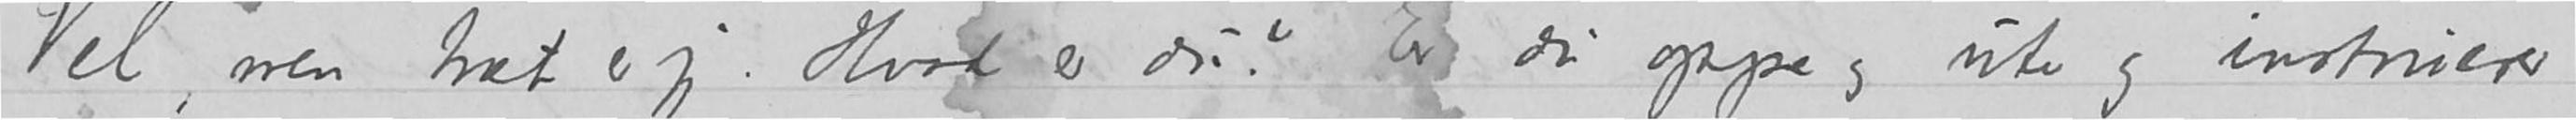Vel, men træt er jeg.  Hvad er du?  Er du oppe og ute og instruerer
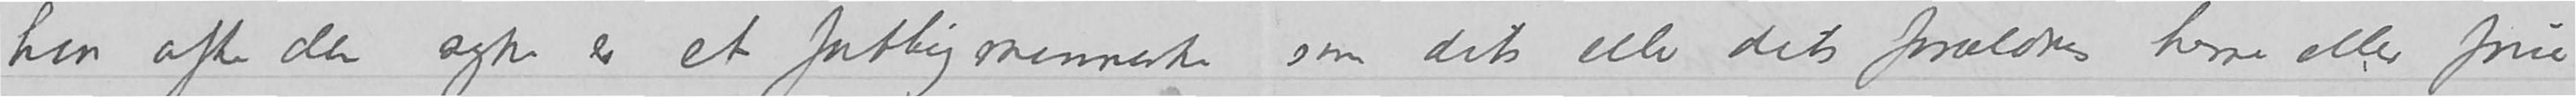hvor ofte den syke er et fattigmenneske som dets eller dets forældres herre eller frue
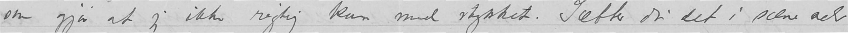som gjør at jeg ikke rigtig kan med stykket. Sætter du det i scene selv
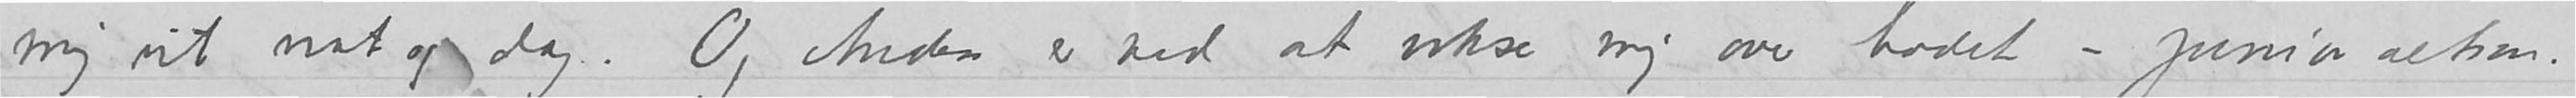mig ut nat og dag. Og Anders er ved at vokse mig over hodet - junior altsaa.
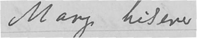Mange hilsener
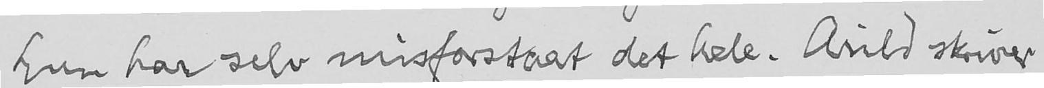hun har selv misforstaat det hele. Arild skriver
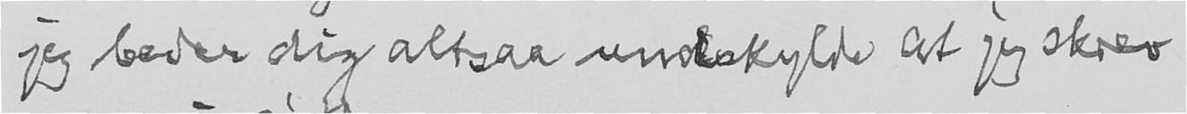jeg beder dig altsaa undskylde at jeg skrev
